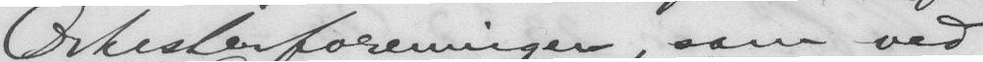Orkesterforeningen, som ved
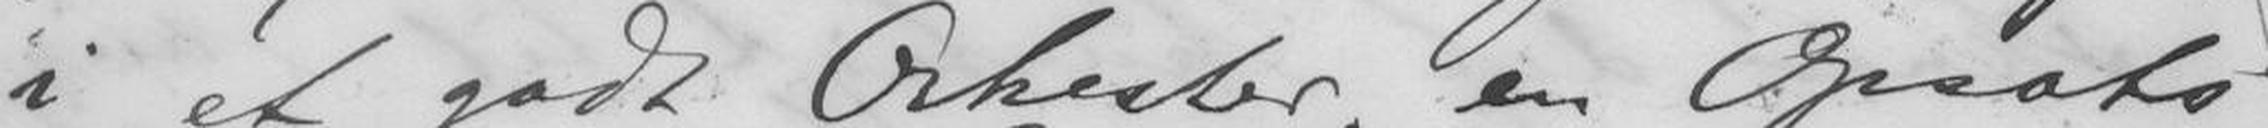i et godt Orkester, en Opsats
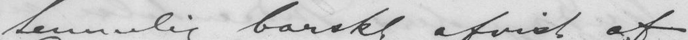temmelig barskt afvist af
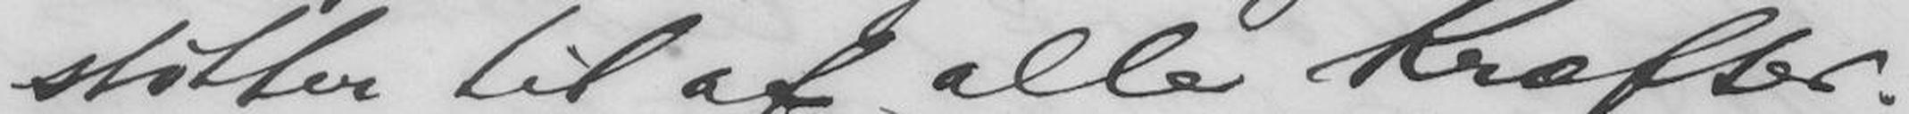støtter til af alle Kræfter.
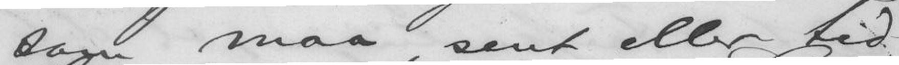som maa, sent eller tid-
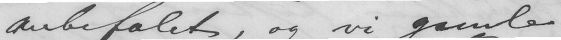anbefalet, og vi gamle
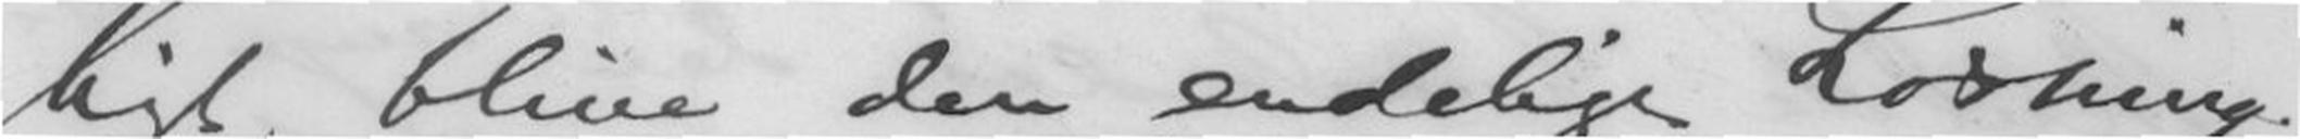ligt, blive den endelige Løsning.
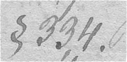§ 334.
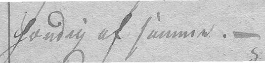hængig af samme. –
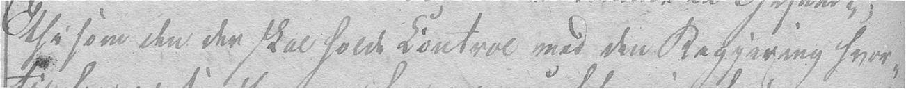thi som den der skal holde Control med den Regjering hvor-
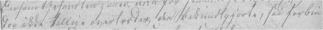dog ikke tillige overtræder den bekjendtgjorte, saa forbin-
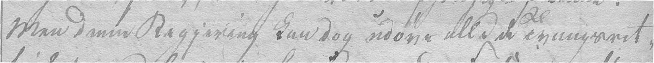Men denne Regjering kan dog udøve alle de Tvangsret-
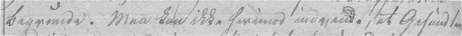begrunde. Man kan ikke herimod indvende, at Gesandten
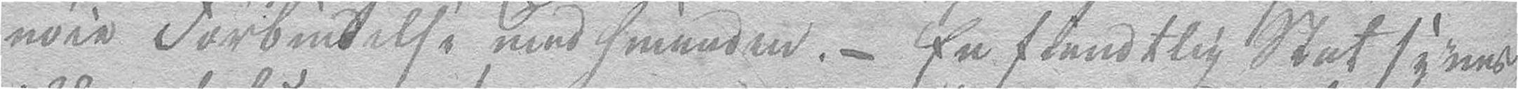nøie Forbindelse med hinanden. – En fiendtlig Stat synes
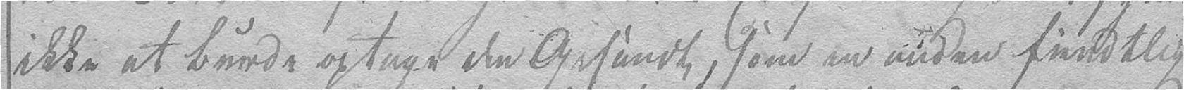ikke at burde optage den Gesandt, som en anden fiendtlig
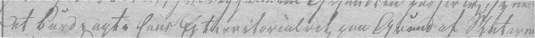at burde agte hans Exterritorialret paa Grund af Staternes
